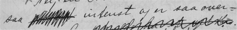saa  intenst og er saa over-
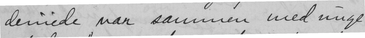dernede var sammen med unge
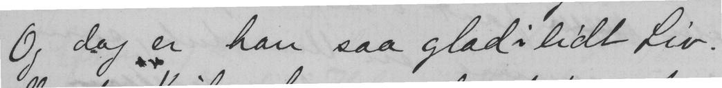Og dog er han saa glad i lidt Liv.
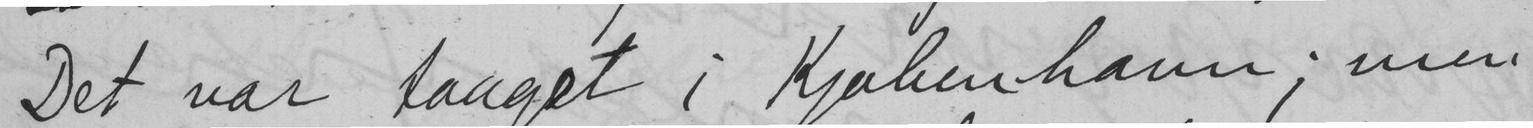Det var taaget i Kjøbenhavn; men
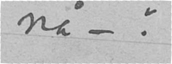nå – ?
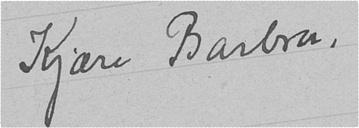Kjære Barbra,
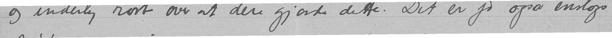og inderlig rørt over at dere gjorde dette. Det er jo også enslags
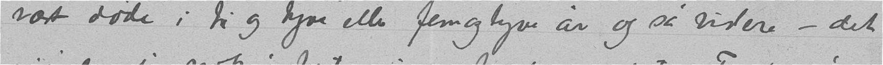vært døde i ti og tyve eller femogtyve år og så videre - det
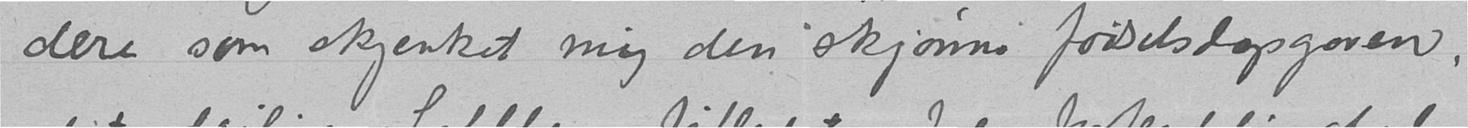dere som skjenket mig den skjønne fødselsdagsgaven,
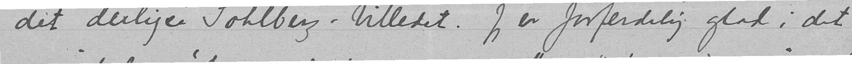det deilige Sohlberg-billedet. Jeg er forferdelig glad i det
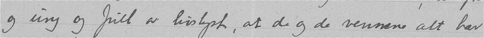og ung og full av livslyst, at de og de vennene alt har
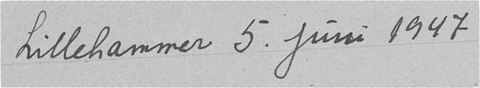Lillehammer 5. juni 1947
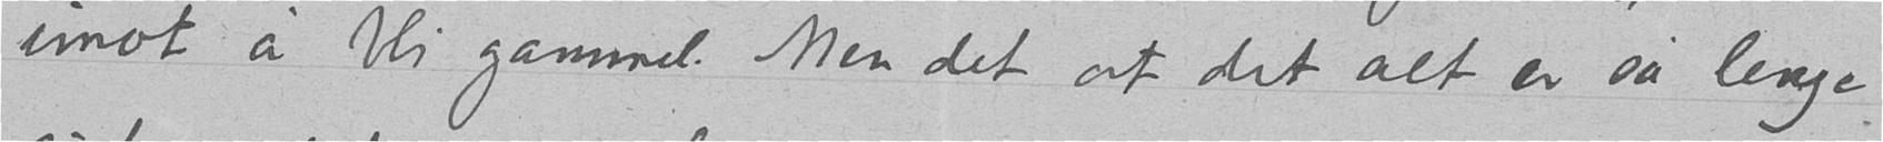imot å bli gammel. Men det at det alt er så lenge
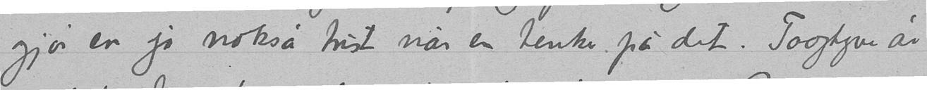gjør en jo nokså trist når en tenker på det. Toogtyve år
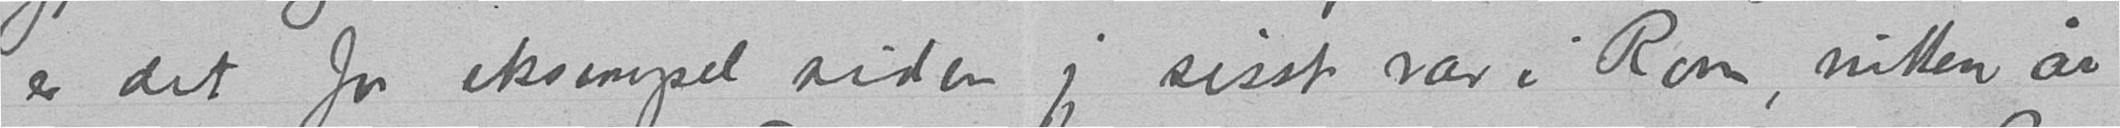er det for eksempel siden jeg sisst var i Rom, nitten år
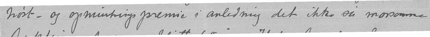trøst – og opmuntringspremie i anledning det ikke så morsomme
